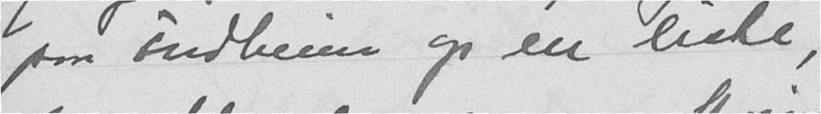paa Fridheim op en liste,
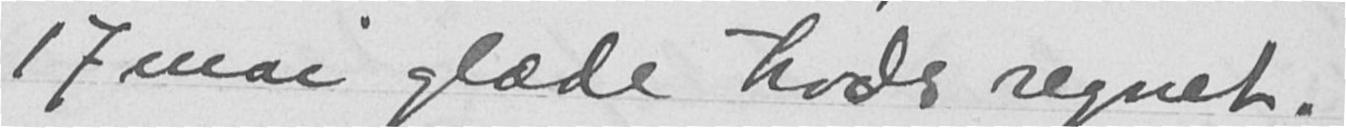17 mai glæde trods regnet.
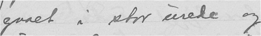gaaet i stor urede og
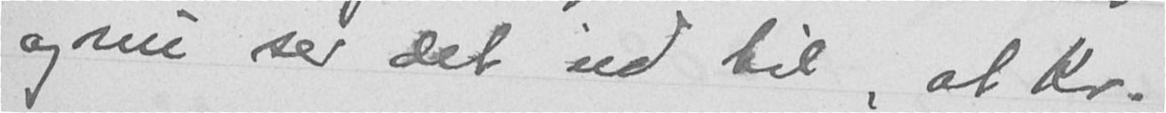og nu ser det ud til, at Kr.
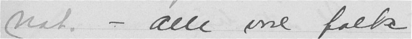nat - alle vore folk
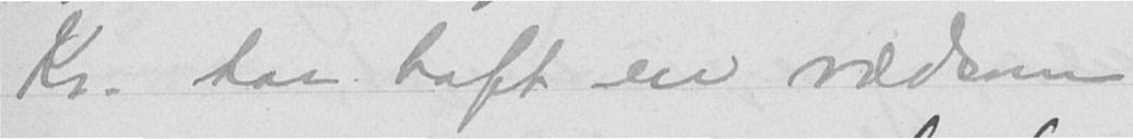Kr. har haft en rædsom
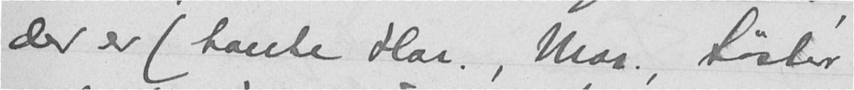der er (tante Har., Mor., Søster
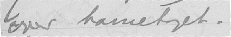over barnetoget.
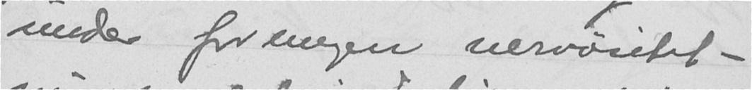under for megen nervøsitet -
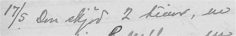17/5 Don skjød 2 tiurer, en
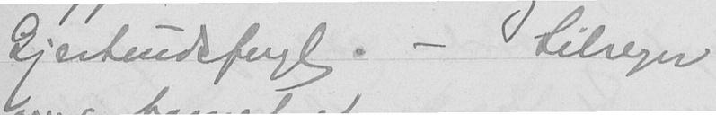Gjertrudsfugl. - Silregn
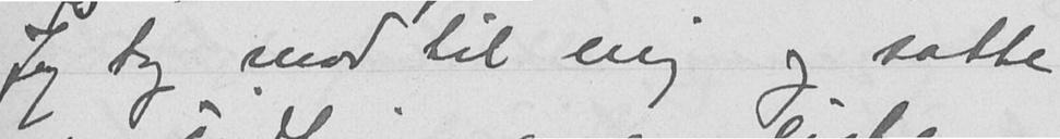Jeg tog mod til mig og satte
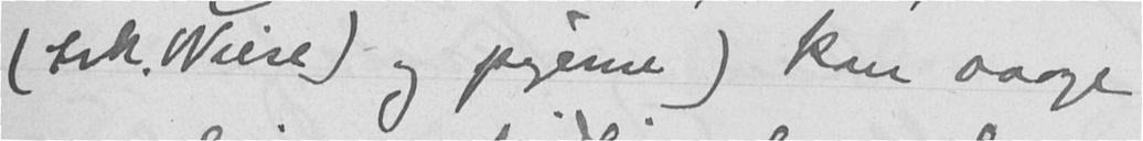(frk. Wiese) og pigerne) kan vaage
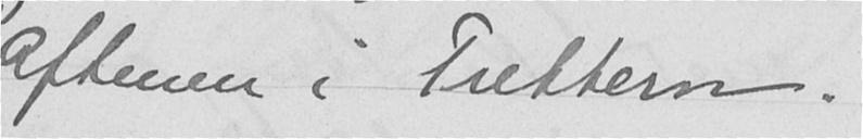aftenen i Trettern.
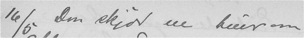16/5 Don skjød en tiur om
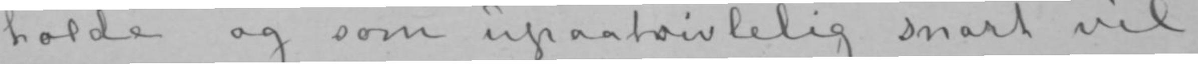holde og som upaatvivlelig snart vil
